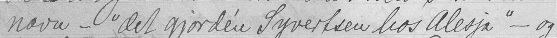navn - "det gjorde'n Syvertsen hos Alesja" - og
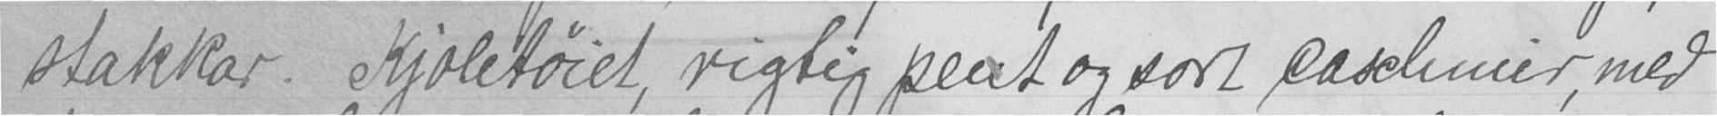stakkar. Kjoletøiet, rigtig pent og sort caschmier, med
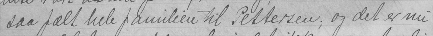saa fælt hele familien til Pettersen; og det er nu
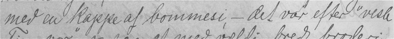med en kappe af bommesi, - det var efter "vesle
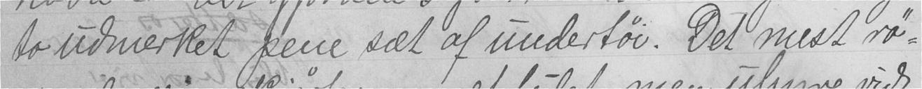to udmerket pene sæt af undertøi. Det mest rø-
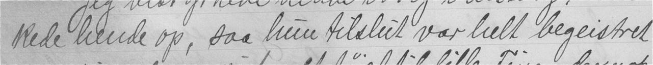kede hende op, saa hun tilslut var helt begeistret
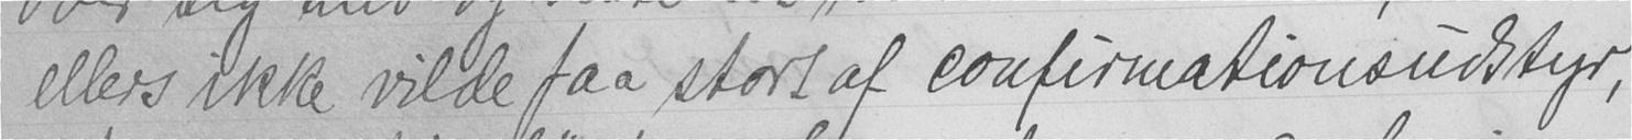ellers ikke vilde faa stort af confirmationsudstyr,
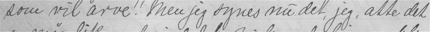som vil arve! Men jeg synes nu det, jeg, atte det
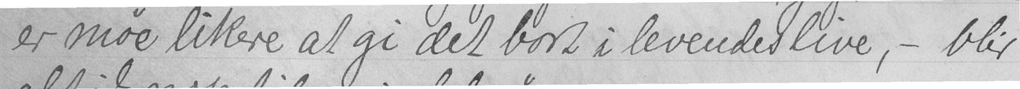er møe likere at gi det bort i levendes live, - blir
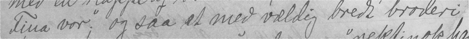Tina vor;" og saa et med vældig bredt broderi
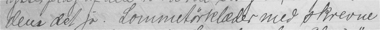dem det jo. Lommetørklæder med skrevne
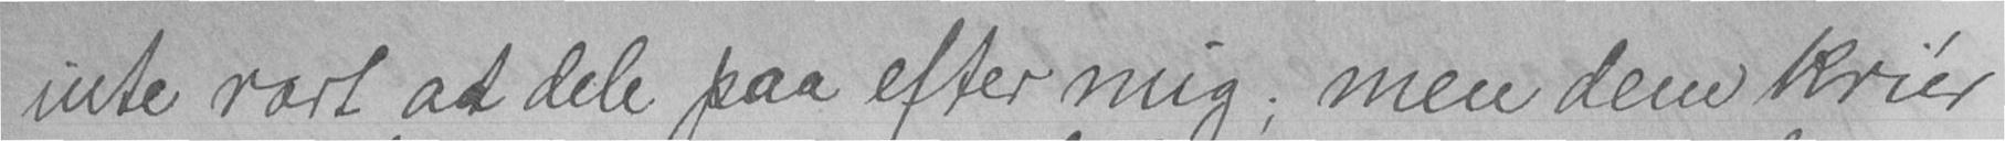inte rart at dele paa efter mig; men dem kri'er
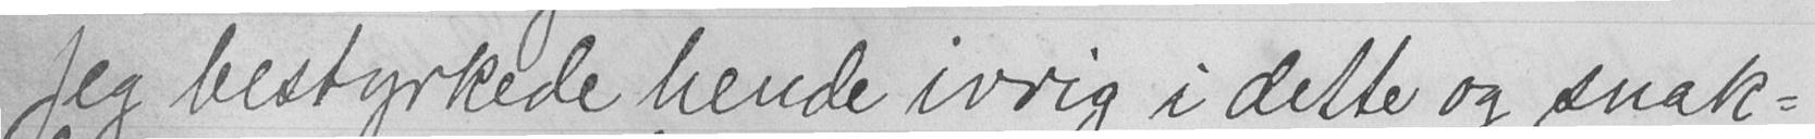Jeg bestyrkede hende ivrig i dette og snak-
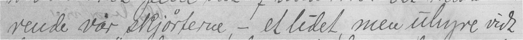rende var skjørterne, - et lidet, men uhyre vidt
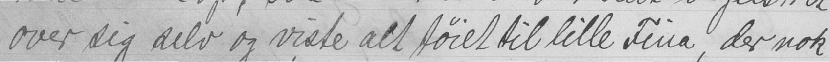over sig selv og viste alt tøiet til lille Tina, der nok
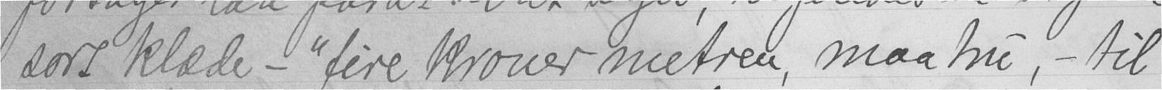sort klæde - "fire kroner metren, maa tru, - til
